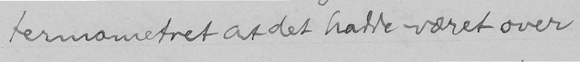termometret at det hadde været over
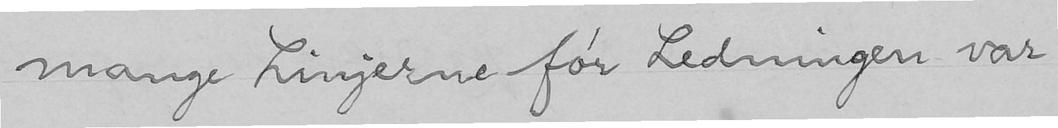mange Linjerne før Ledningen var
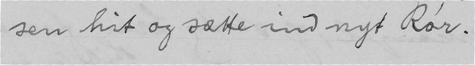sen hit og sætte ind nyt Rør.
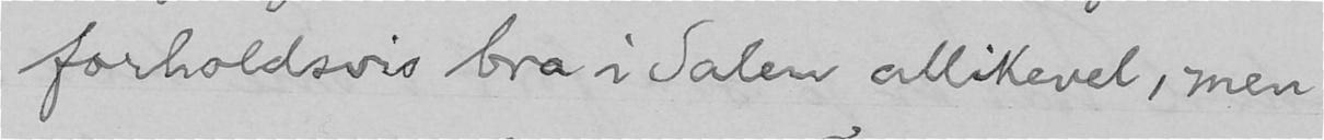forholdsvis bra i Salen allikevel, men
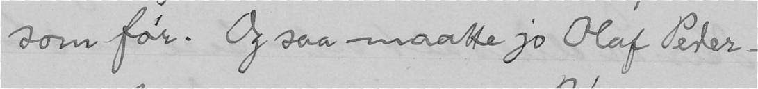som før. Og saa maatte jo Olaf Peter-
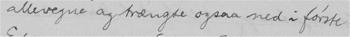allevegne og trængte ogsaa ned i første
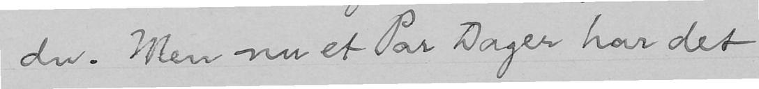du. Men nu et Par Dager har det
